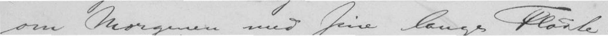om Morgenen med sine lange Fløite-
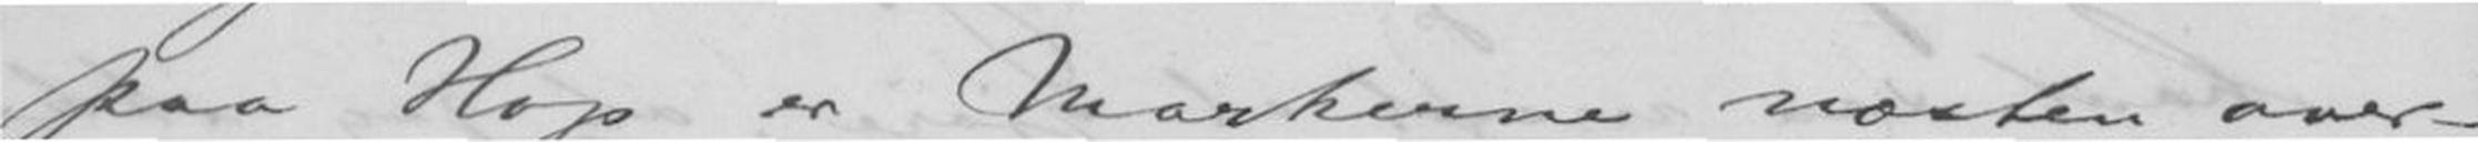paa Hop er Markerne næsten over-
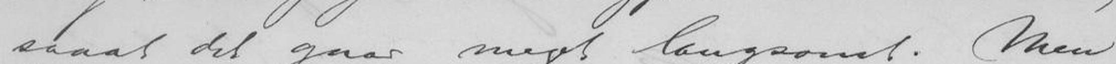saaat det gaar meget langsomt. Men
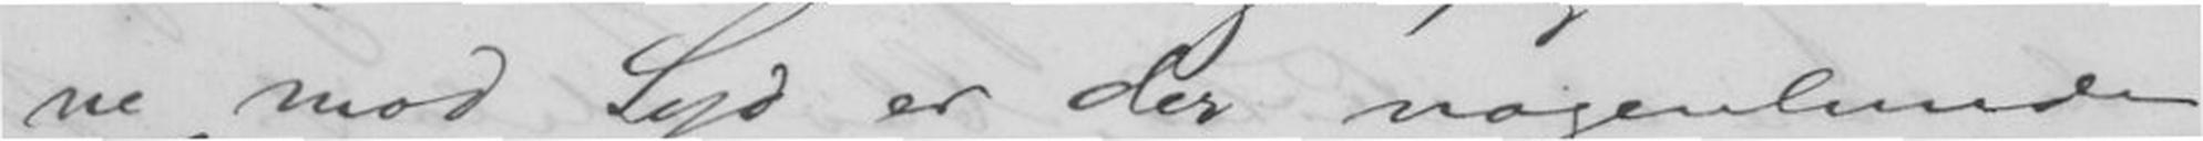ne mod Syd er der nogenlunde
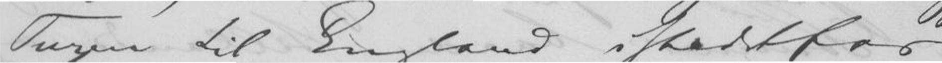Turen til England istedetfor
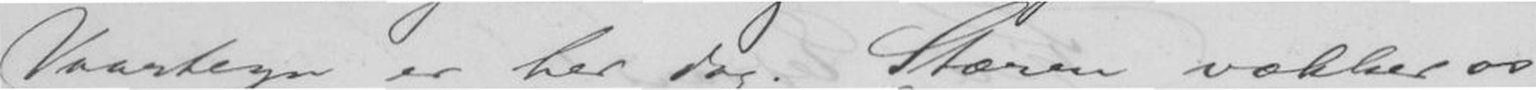Vaartegn er her dog. Stæren vækker os
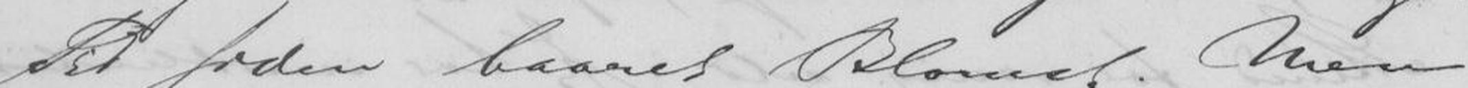Tid siden baaret Blomst. Men
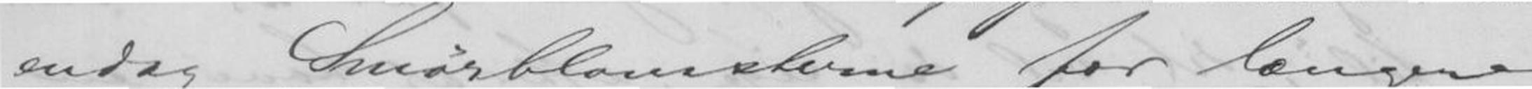endog Smørblomsterne for længere
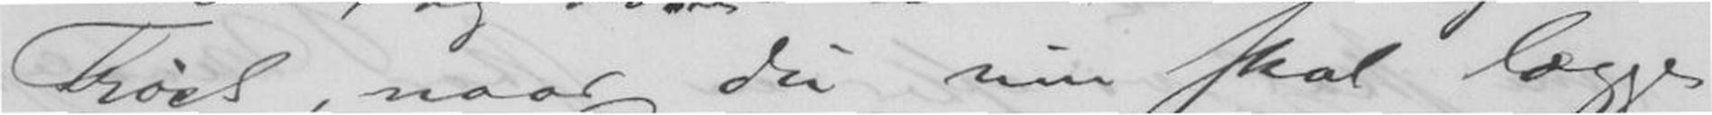Trøst, naar Du nu skal lægge
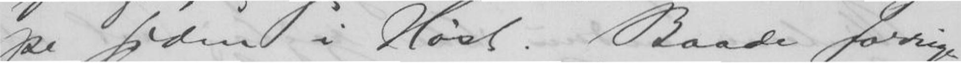pe siden i Høst. Baade forrige
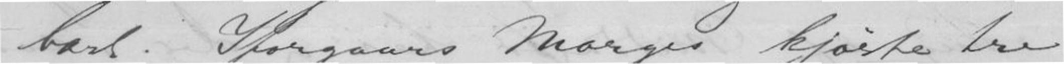bart. Iforgaars Morges kjørte tre
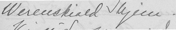Werenskiold hjem.
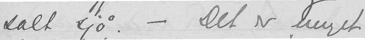salt sjø. - Det er meget
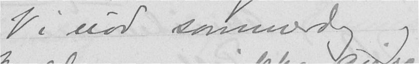Vi nød sommerdag og
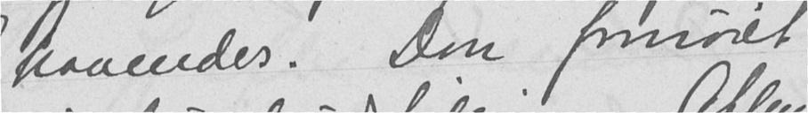havender. Don fornøiet
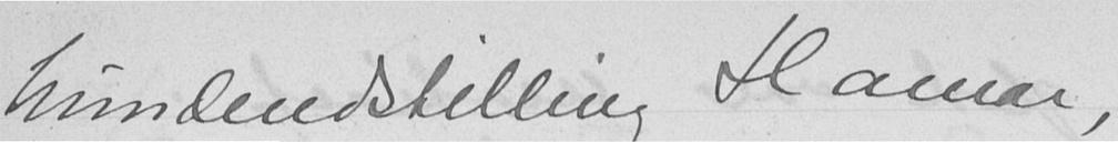hundeudstilling Hamar,
